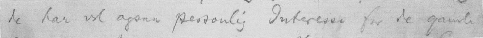de har vel ogsaa personlig Interesse for de gamle
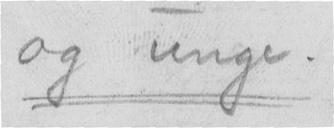og unge.
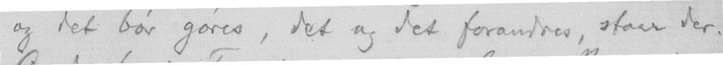og det bør gøres, det og det forandres, staar der.
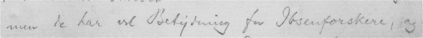men de har vel Betydning for Ibsenforskere, og
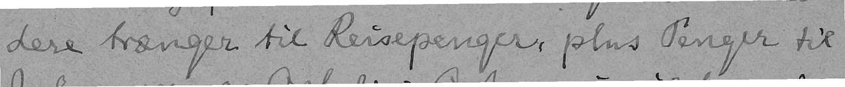dere trænger til Reisepenger, plus Penger til
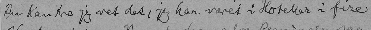Du kan tro jeg vet det, jeg har været i Hoteller i fire
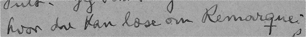hvor du kan læse om Remarque.
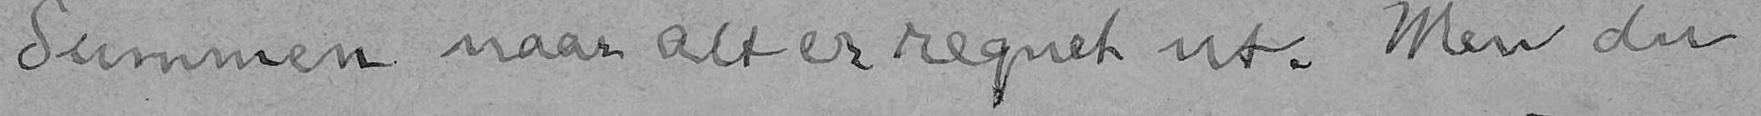Summen naar alt er regnet ut. Men du
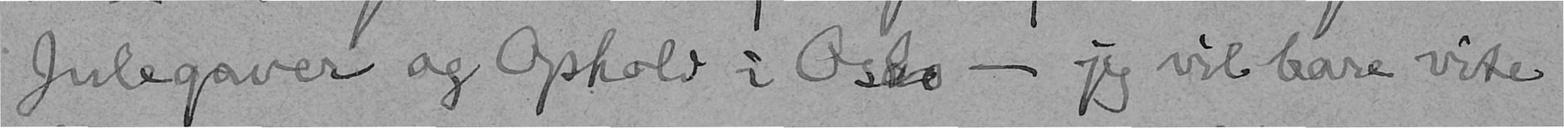Julegaver og Ophold i Oslo - jeg vil bare vite
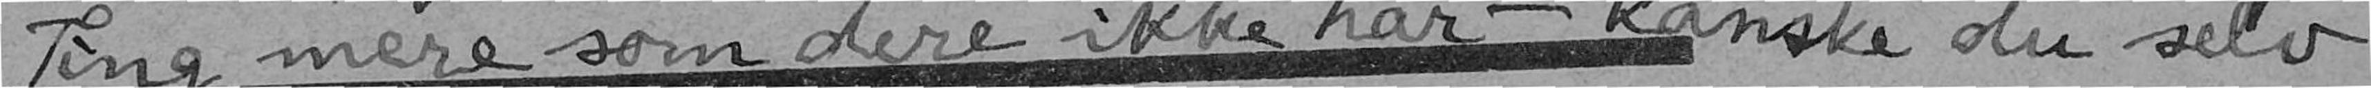Ting mere som dere ikke har - kanske du selv
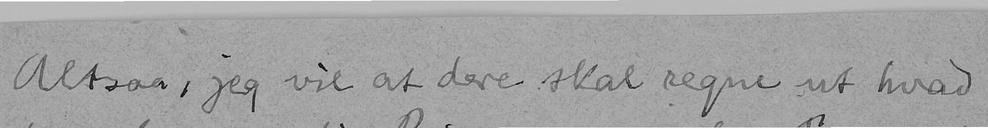Altsaa, jeg vil at dere skal regne ut hvad
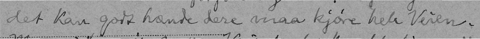det kan godt hænde dere maa kjøre hele Veien.
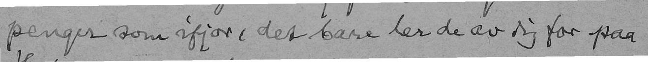penger som ifjor, det bare ler de av dig for paa
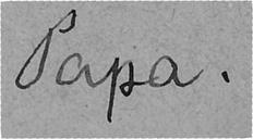Papa.
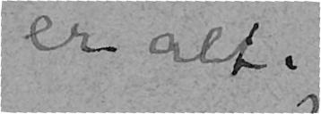er alt.
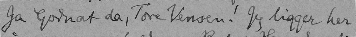Ja Godnat da, Tore Vensen! Jeg ligger her
